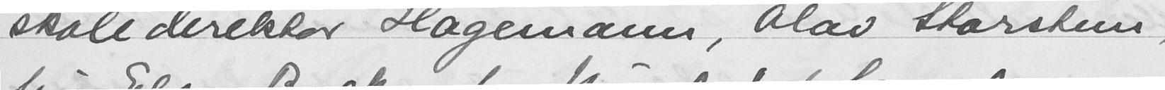skoledirektor Hagemann, Olav Storstein,
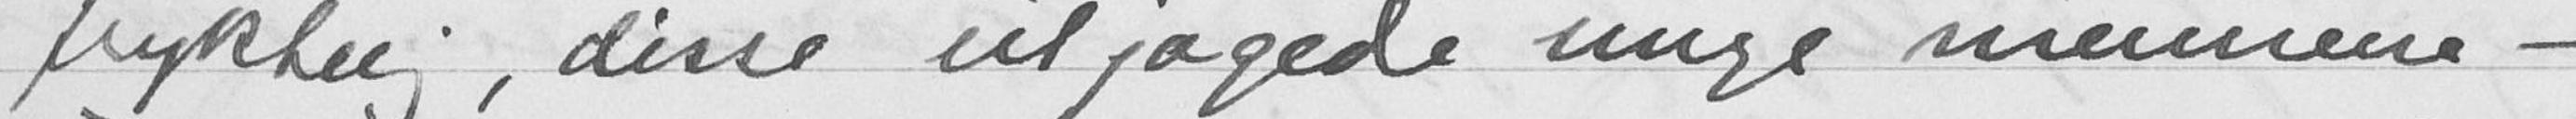fryktelig, disse utjagede unge mennene -
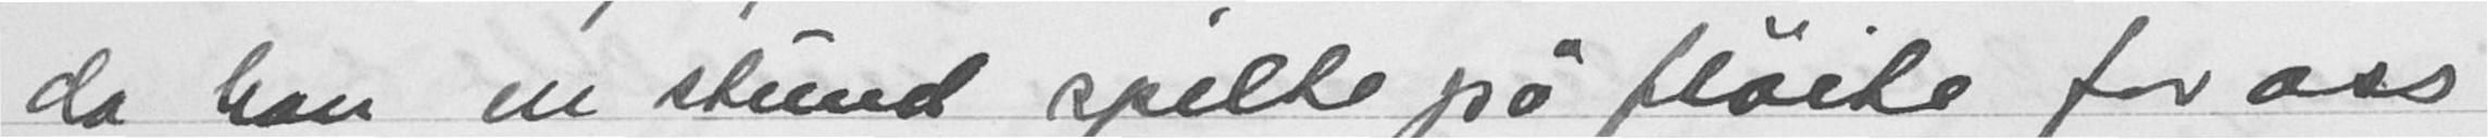da han en stund spilte på fløite for oss
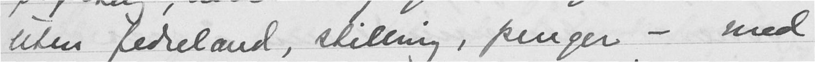uten fedreland, stilling, penger - med
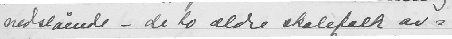nedslående - de to ældre skolefolk av-
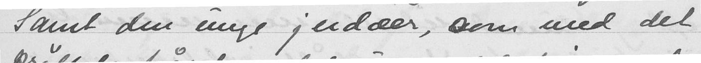Samt den unge judæer, som med det
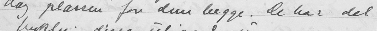dag plassen for dem begge. De har det
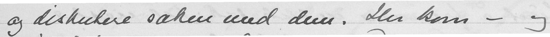og diskutere saken med dem. Her kom - og
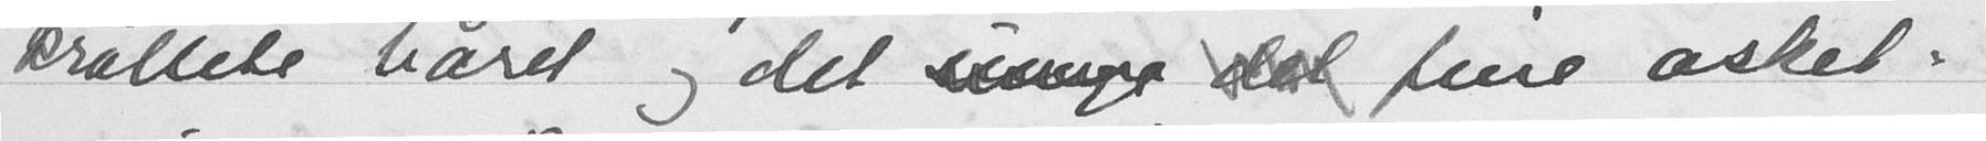krøllete håret og det  fine asket-
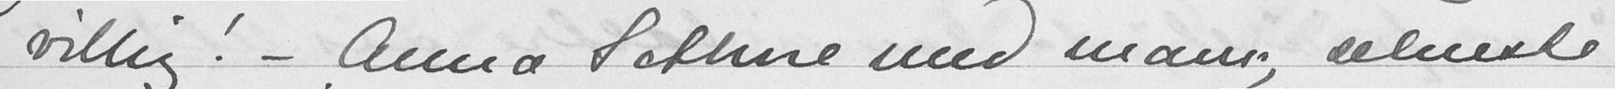villig! - Anna Sethne med mann, selveste
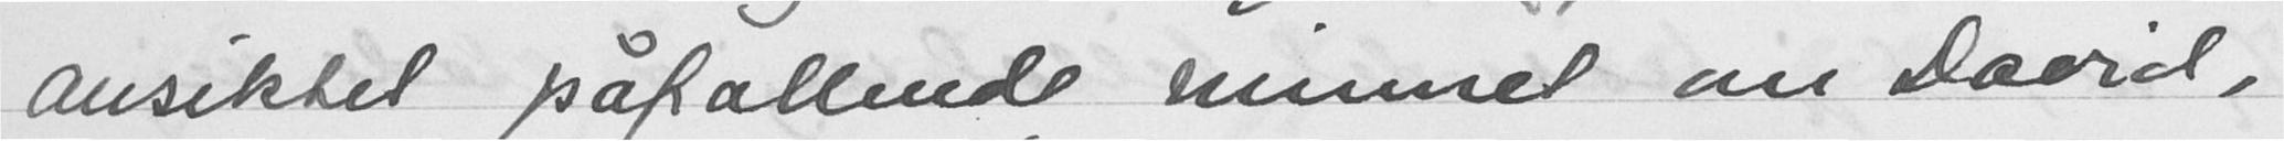ansiktet påfallende minnet om David,
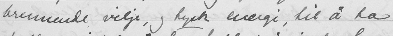brennende vilje, og tysk energi, til å ta
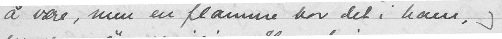å være, men en flamme bor det i ham, og
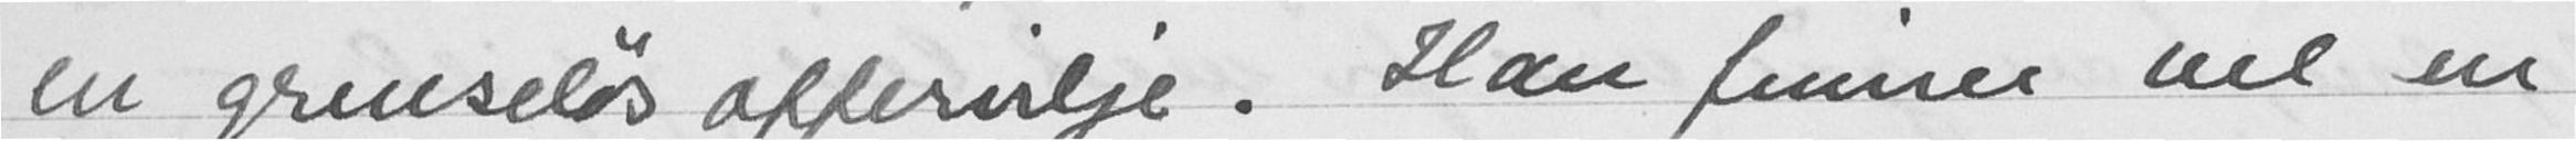en grenseløs offervilje. Han finner vel en
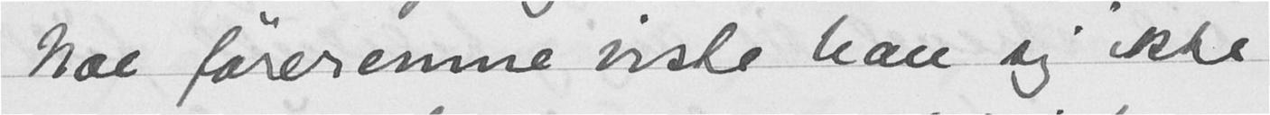Noe føreremne viste han sig ikke
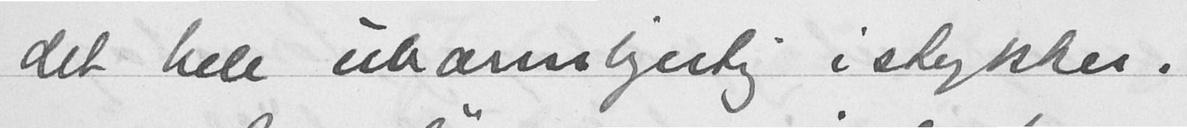det hele ubarmhjertig i stykker.
